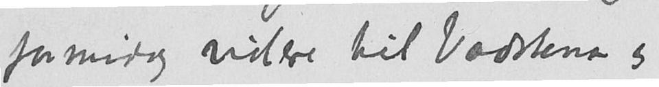formidag videre til Vadstena og
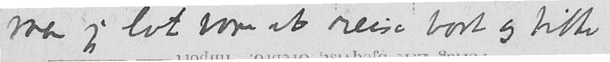men jeg lot være at reise bort og titte
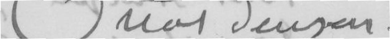Otto Benzon
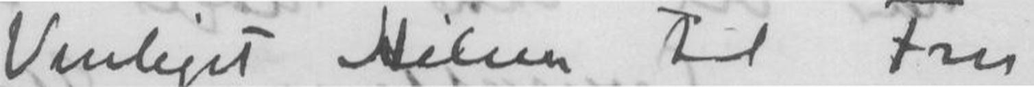Venligst Hilsen til Fru
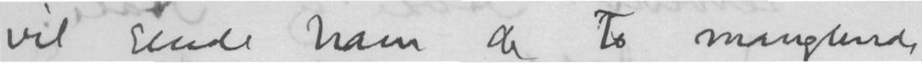vil sende ham de to manglende
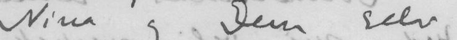Nina og Dem selv.
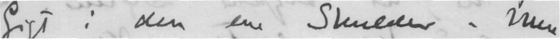Gigt i den ene Skulder. Men
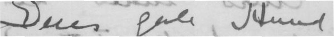Deres gode Hund
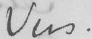Vers.
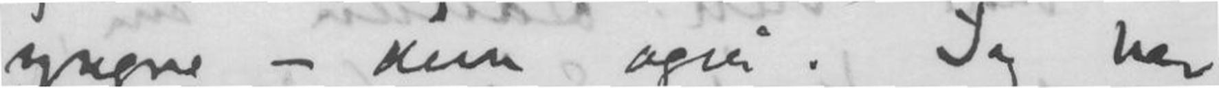yngre - kun også. Jeg har
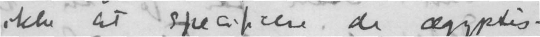ikke at specificere de ægyptis-
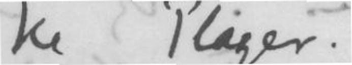ke Plager.
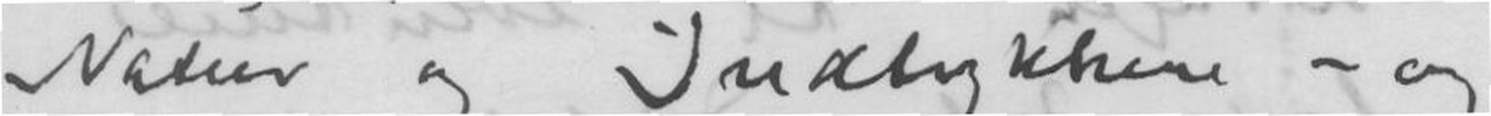Natur og Indtrykkene - og
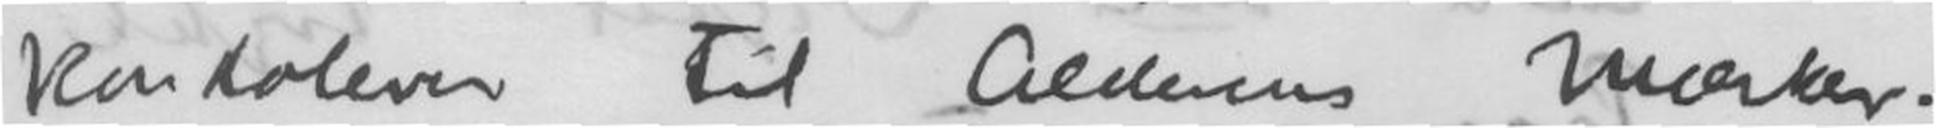kondolerer til Alderens Mærker.
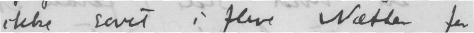ikke sovet i flere Nætter for
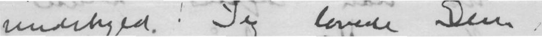undskyld! Jeg lovede Dem
